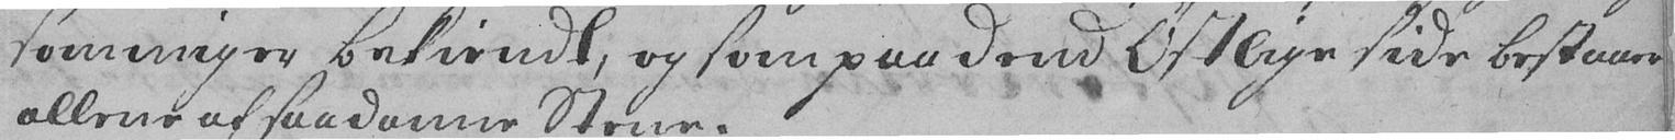som mig er bekiendt, og som paa dend østlige side bestaaer
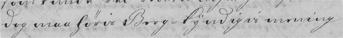dog maa høris Berg-kyndigis mening
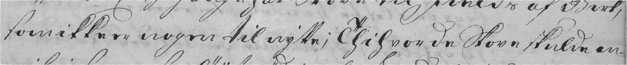som ikke er nogen til nytte; Thi hvor de Skove skulde an-
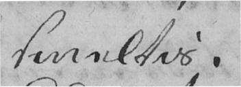smeltis.
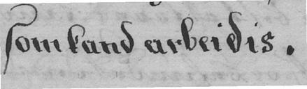som kand arbeidis.
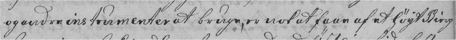og andre instrumenter at bruge, er nok at faae af et høyt Bierg
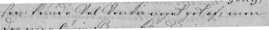som kunde Vel Ventis noget got af, men
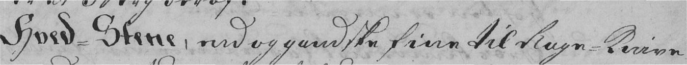Hved-Stene, end og gandske fine til Rage-Knive
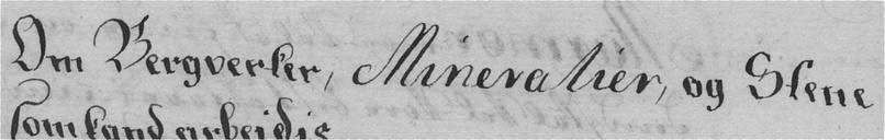Om Bergverker, Mineralier, og Stene
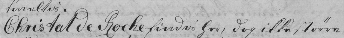Christal de Roche findis her, dog ikke større
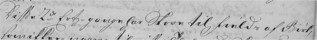Disse 2de Ertz-gange har Skove til Fields af Birk,
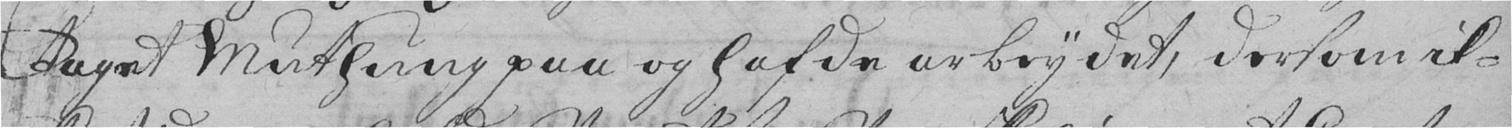taget Muthung paa og hafde arbeydet, dersom ik-
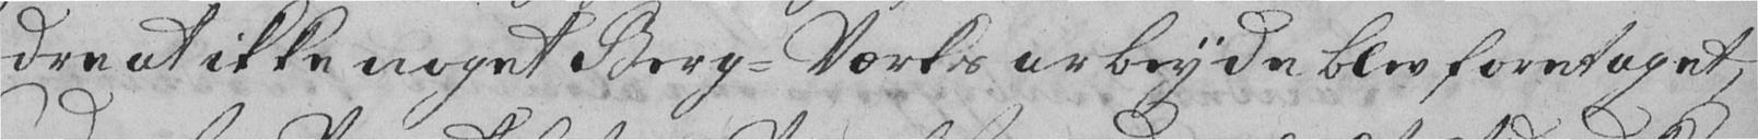dre at ikke noget Berg-Værks arbeyde blev foretaget,
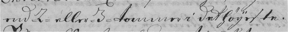end 2 eller 3 tommer i det høyeste.
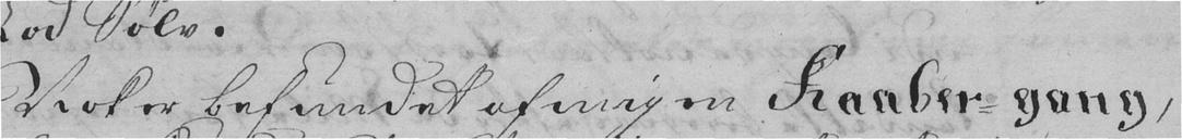Nok er befundet af mig en Kaaber-gang,
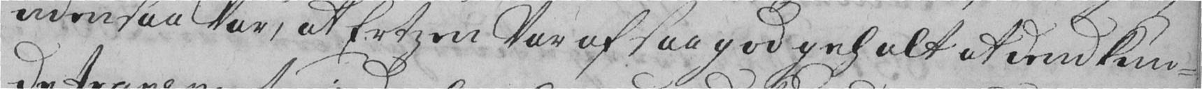uden saa Var, at Ertzen Var af saa god gehalt at dend kun-
